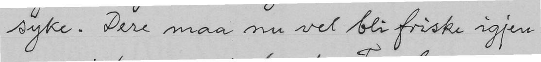syke. Dere maa nu vel bli friske igjen
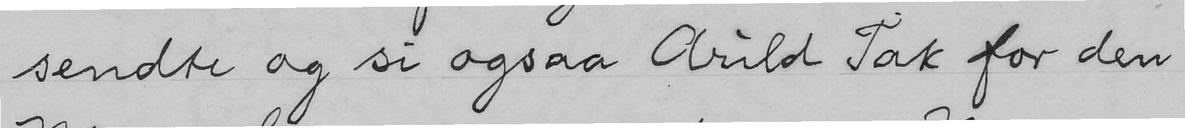sendte og si ogsaa Arild Tak for den
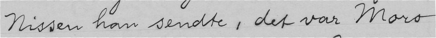Nissen han sendte, det var Moro
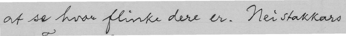at se hvor flinke dere er. Nei stakkars
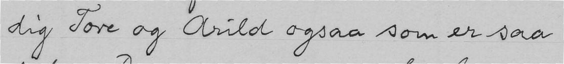dig Tore og Arild ogsaa som er saa
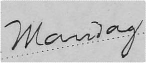Mandag
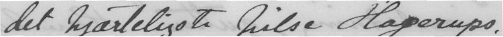det hjærteligste hilse Hagerups,
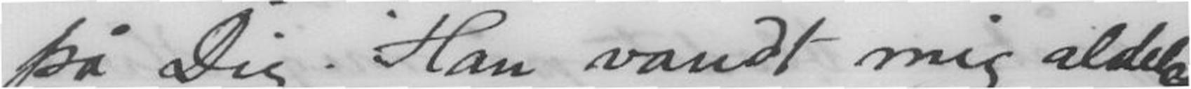på Dig. Han vandt mig aldeles,
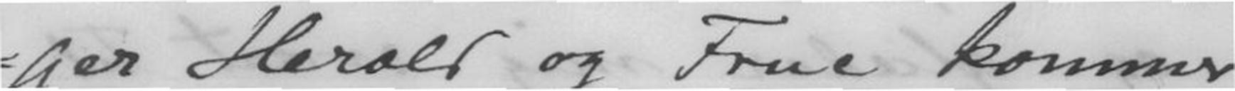ger Herold og Frue kommer
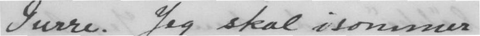Gurre. Jeg skal isommer
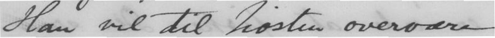Han vil til høsten overvære
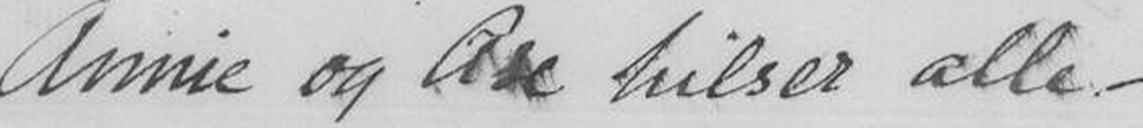Annie og Åse hilser alle.
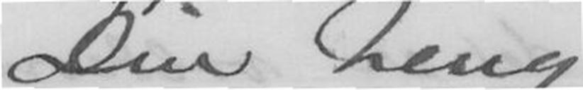Din heng
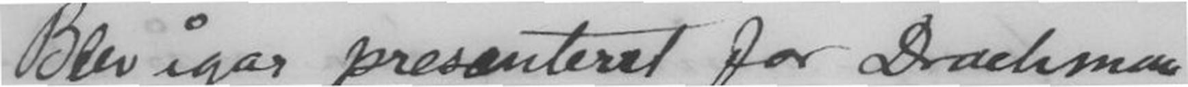Blev igår presenteret for Drachman.
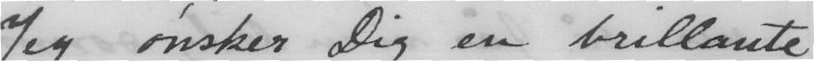Jeg ønsker Dig en brillante
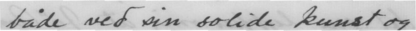både ved sin solide kunst og
